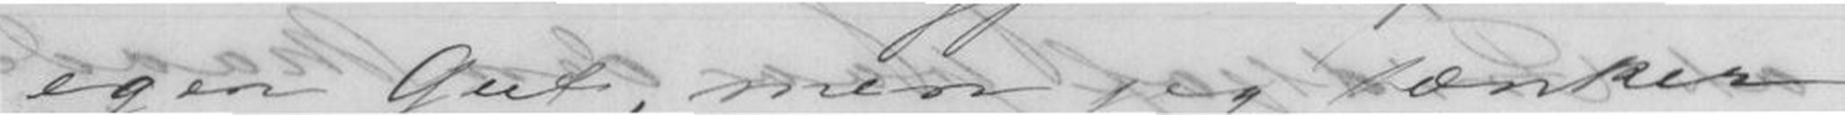egen Gut, men jeg tænker
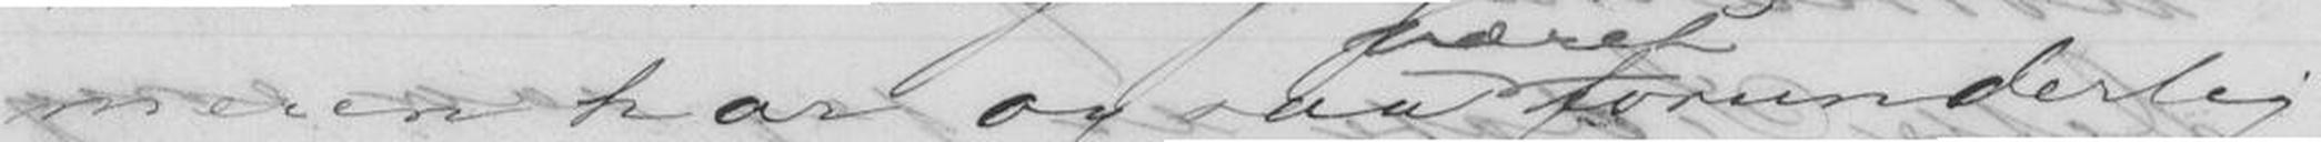meren har ogsaa forunderlig
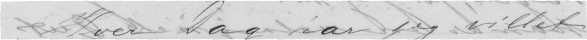Hver Dag har jeg villet
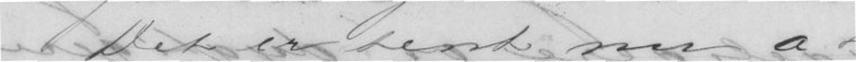Det er sent nu at
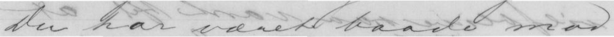Du har været baade mod
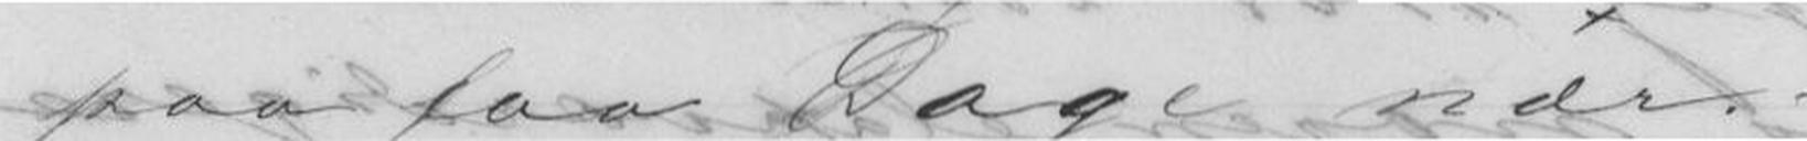paa faa Dage nær.-
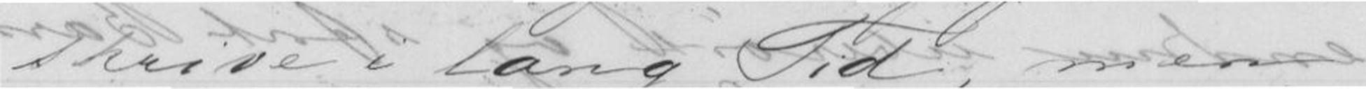skrive i lang Tid, men
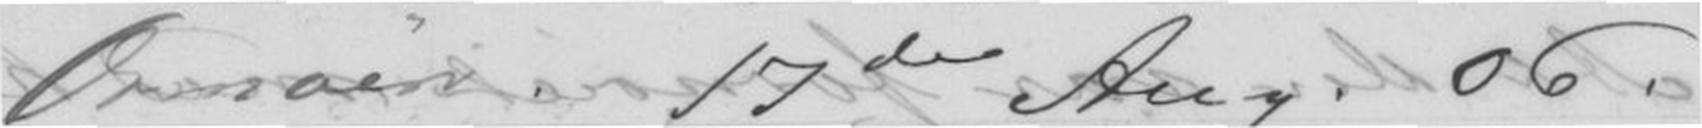Ormøen 17de Aug. 06.
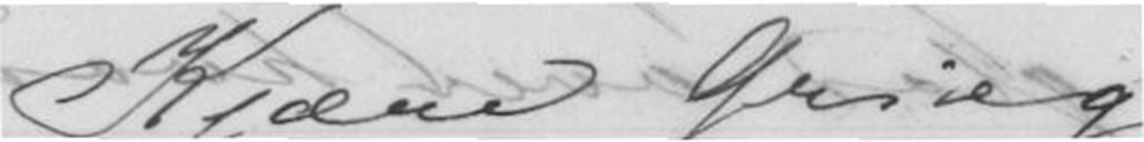Kjære Grieg,
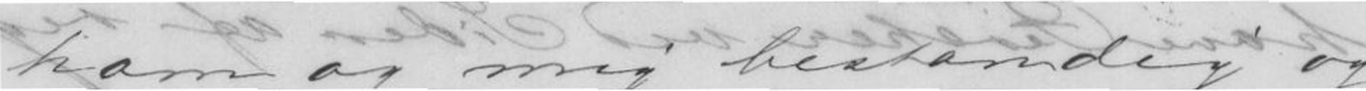ham og mig bestandig og
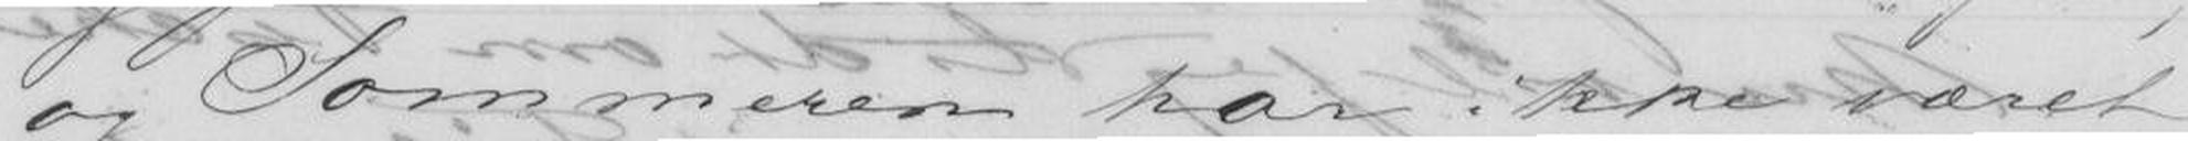og Sommeren har ikke været
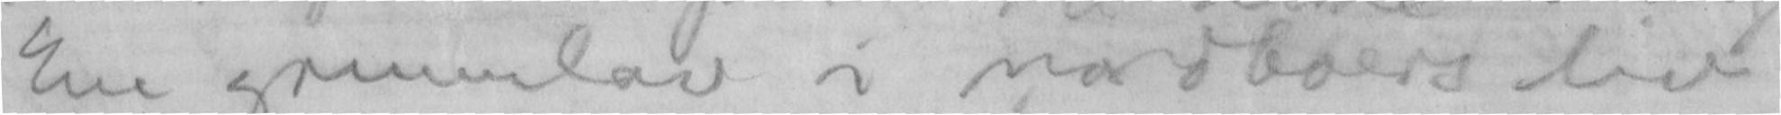Ein grunnlov i nordboers liv
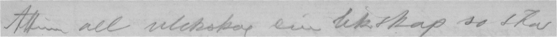Allum all ulikskap ein likskap so stor
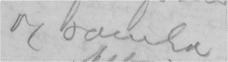og samla.
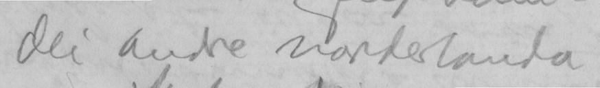dei andre norderlanda.
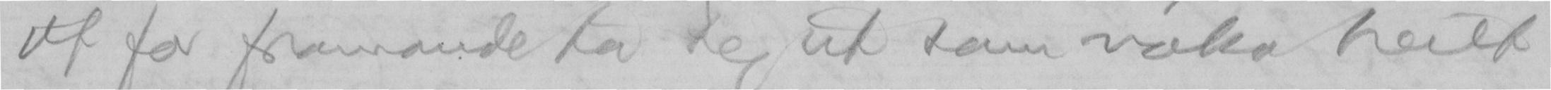at for framande ta seg ut som noko heilt
